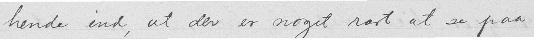hende ind, at der er noget rart at se paa
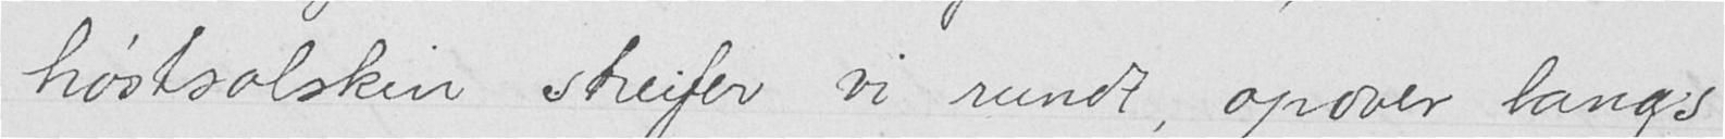høstsolskin streifer vi rundt, opover langs
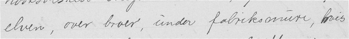elven, over broer, under fabriksmure, hvis
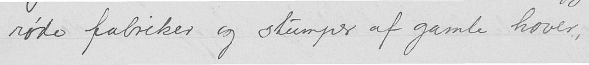røde fabriker og stumper af gamle haver,
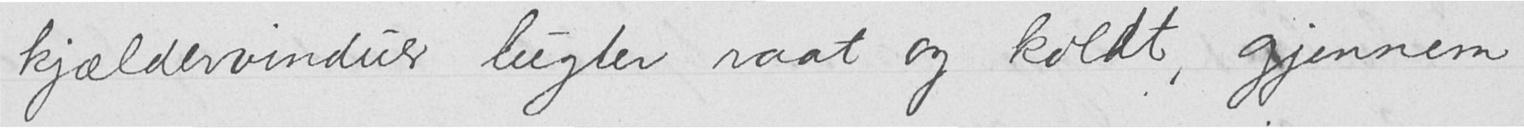kjældervinduer lugter raat og koldt, gjennem
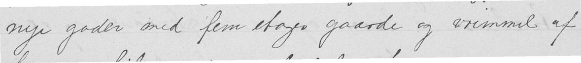nye gader med fem etages gaarde og vrimmel af
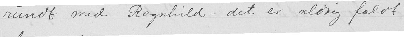rundt med Ragnhild — det er aldrig faldt
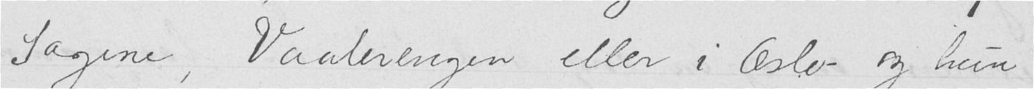Sagene, Vaalerengen eller i Oslo og hun
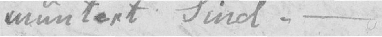muntert Sind. –
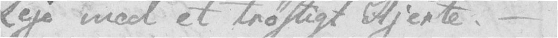Leje med et trøstigt Hjerte. –
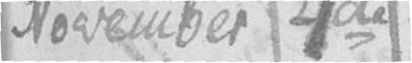November 4de
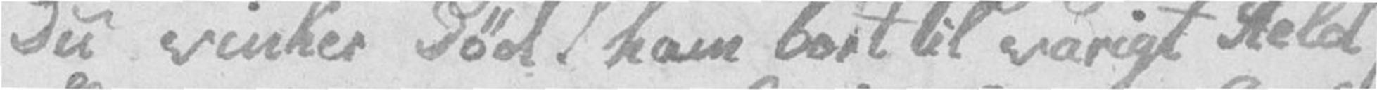Du vinker Død! ham bort til varigt Held,
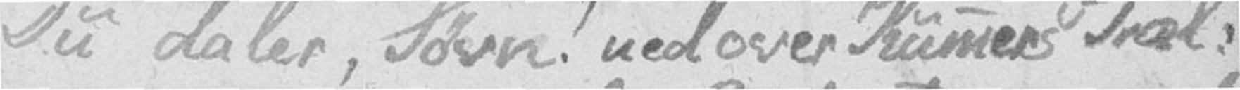Du daler, Søvn! ned over Kummers Træl,
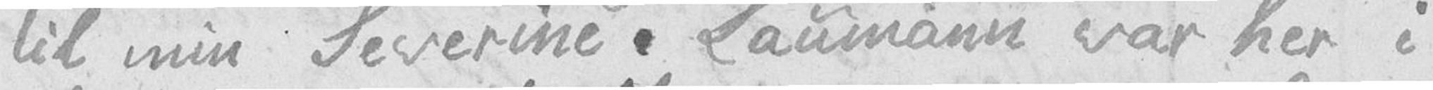til min Severine. Laumann var her i
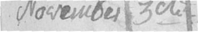November 3de
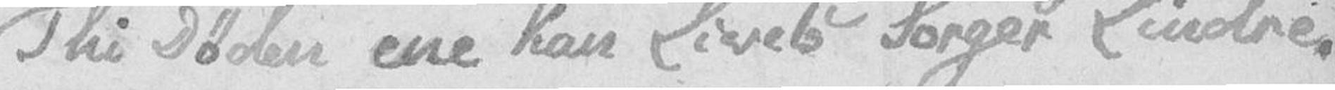Thi Døden ene kan Livets Sorger Lindre.
